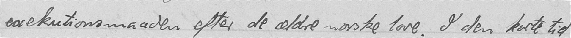exekutionsmaaden efter de ældre norske love. I den korte tid
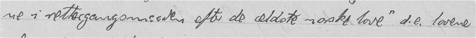ne i rettergangsmaaden efter de ældste norske love" d.e. lovene
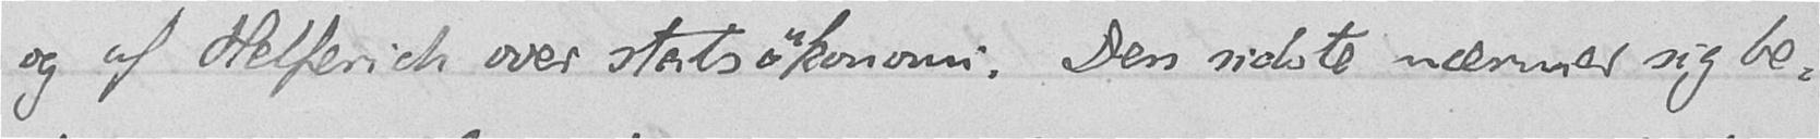og af Helferich over statsøkonomi. Den sidste nærmer sig be-
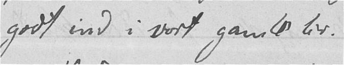godt ind i vort gamle liv.
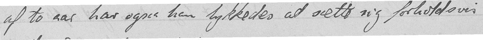af to aar har ogsaa han lykkedes at sætte sig forholdsvis
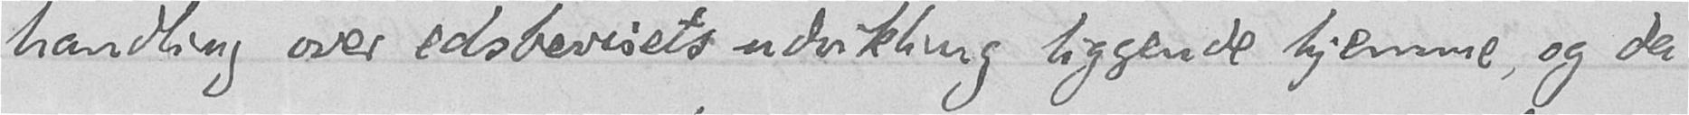handling over edsbevisets udvikling liggende hjemme, og da
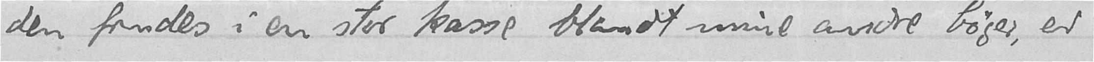den findes i en stor kasse blandt mine andre bøger, er
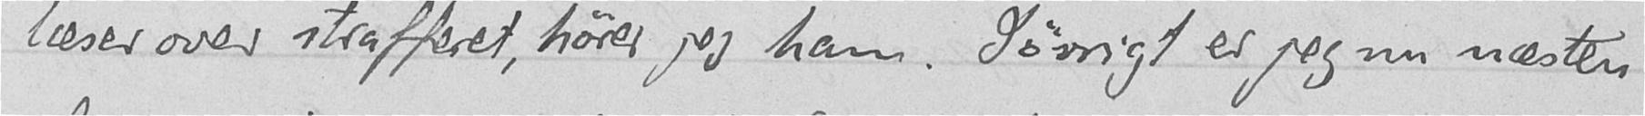læser over strafferet, hører jeg ham. Iøvrigt er jeg nu næsten
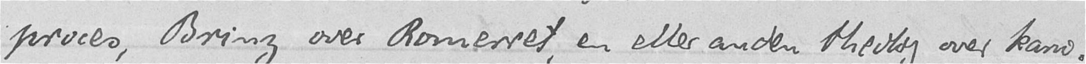proces, Brinz over Romerret, en eller anden theolog over kano-
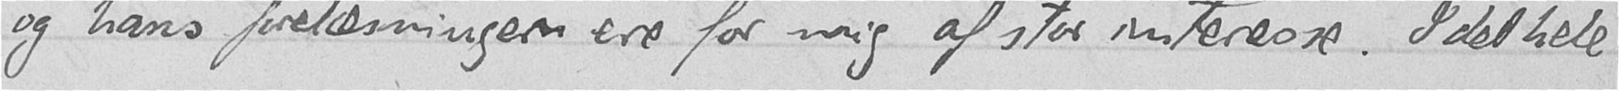og hans forelæsninger ere for mig af stor interesse. I det hele
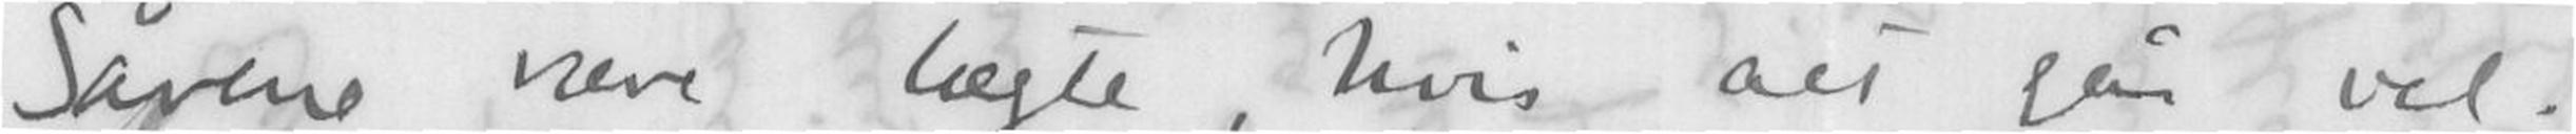Sårene være lægte, hvis alt går vel.
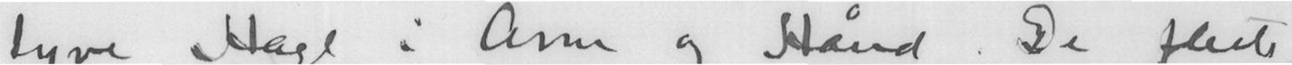tyve Hagl i Arm og Hånd. De fleste
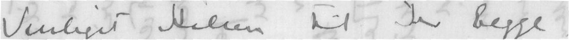Venligst Hilsen til Jeg begge.
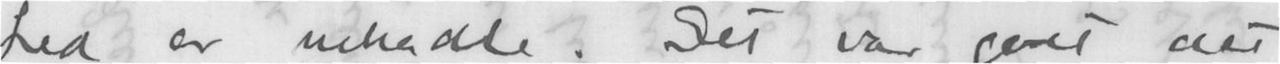Led er uskadte. Det var godt alt
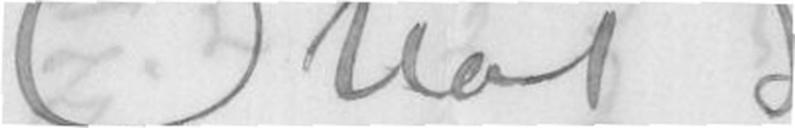Otto B
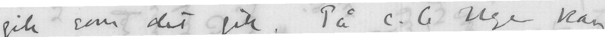gik som det gik. På c. 6 Uge kan
